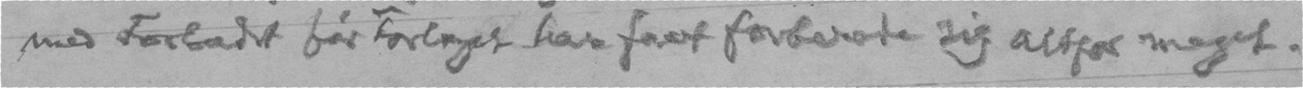med Forbudet før Forlaget har faat forberede sig altfor meget.
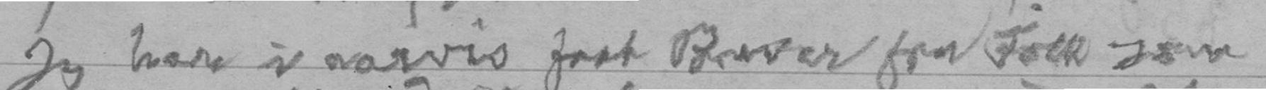Jeg har i aarvis faat Brever fra Folk som
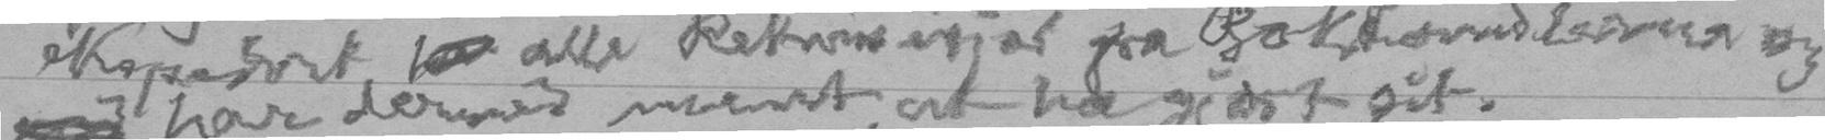ekspedert ha alle Rekvisisjoner fra fra Bokharndlerene og
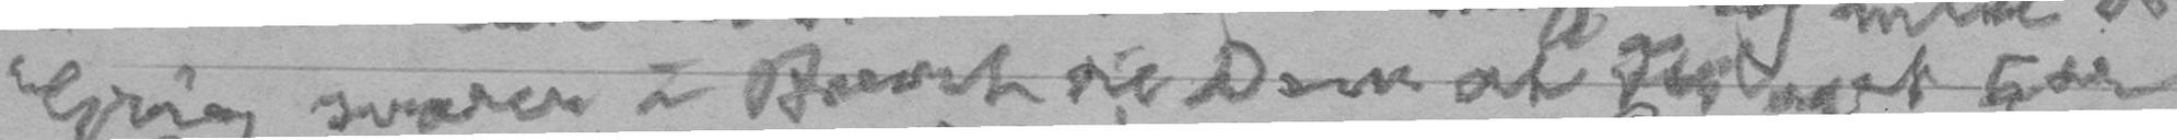Griag svarer i Brevet til Dem at Forlaget har
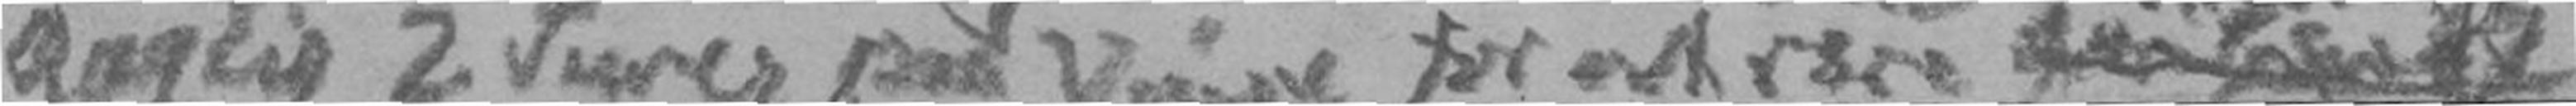daglig 2 Turer paa Veiene for at røre
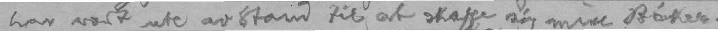har vært ute av Stand til at skaffe sig mine Bøker.
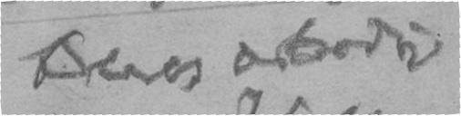Deres ærbødige
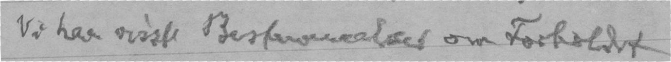Vi har visste Bestemmelser om Forholdet
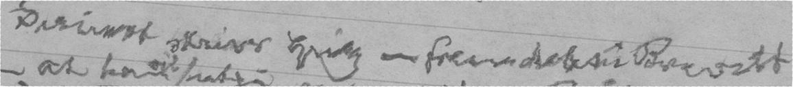Derimot skriver Grieg - fremdeles i Brevett
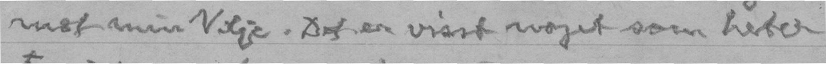mot min Vilje. Det er visst noget som heter
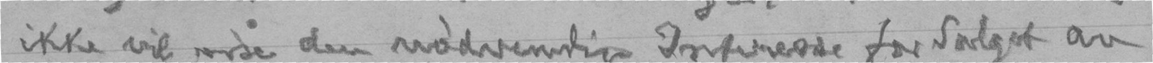ikke vil vise den nødvendige Interesse for Salget av
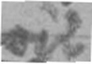vil
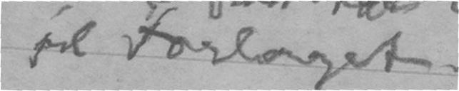til Forlaget.
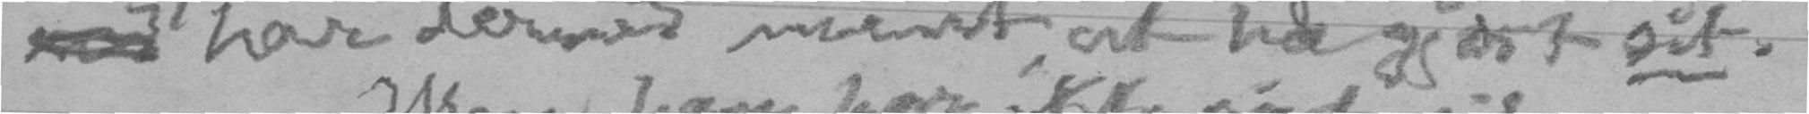vd har dermed ment at ha gjort sit.
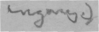engang.)
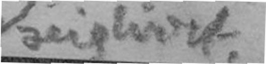seiglivet.
